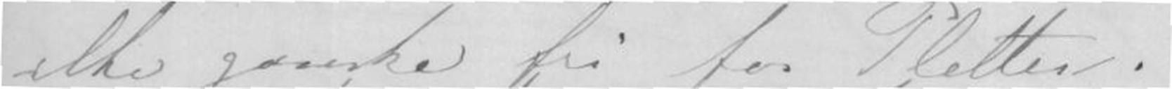ikke ganske fri for Pletter.
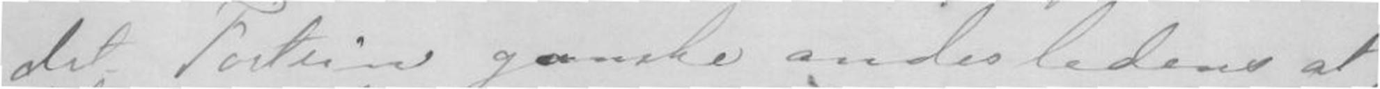det Fortun ganske anderledens at
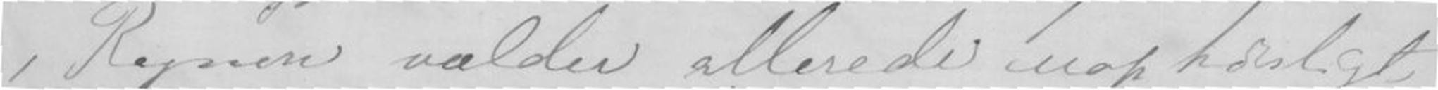,Rigmor valder allerede
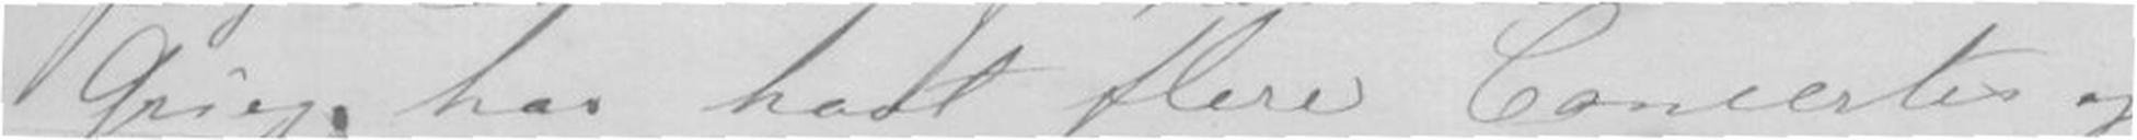Grieg, har hadt flere Concerter og
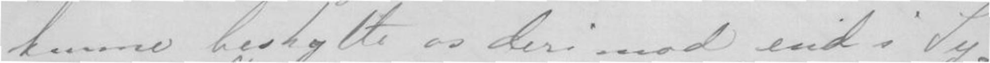kunne beskytte os derimod end i Sy-
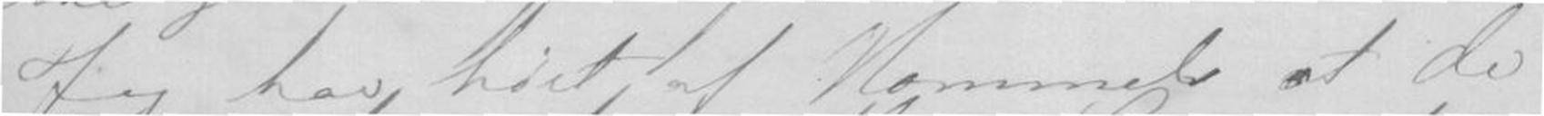Jeg har hørt af Hommels at de
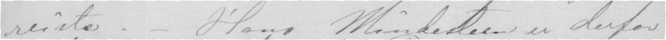reiste.- Hans Mindesleen er derfor
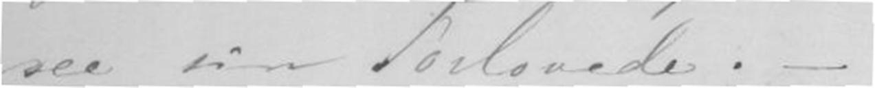see sin Forlovede.-
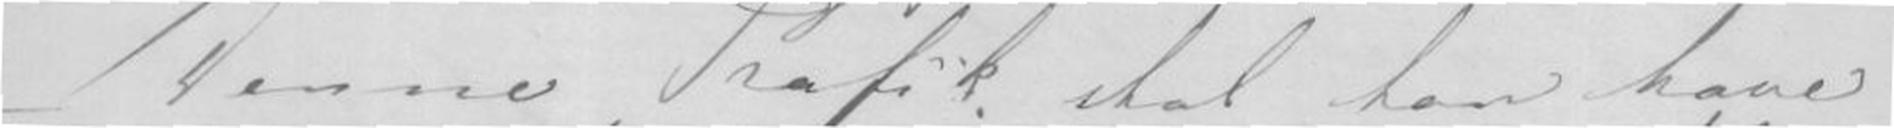Denne Trafik skal han have
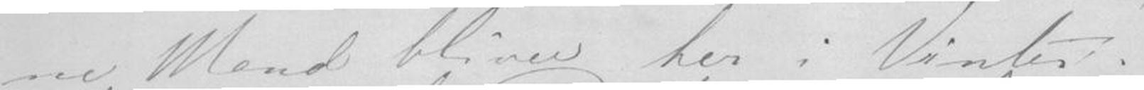ne Mand bliver her i Vinter.
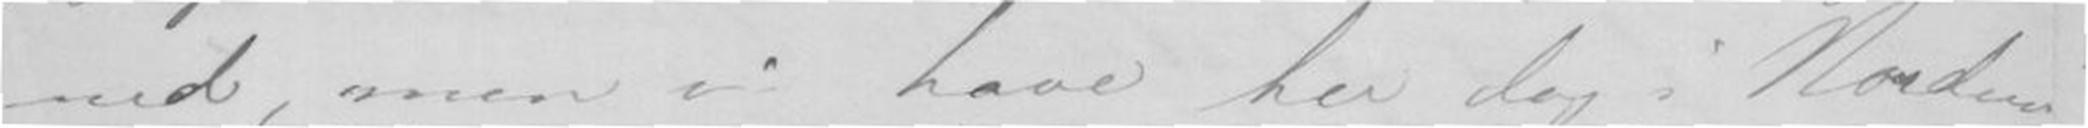med, men vi have her dog i Norden
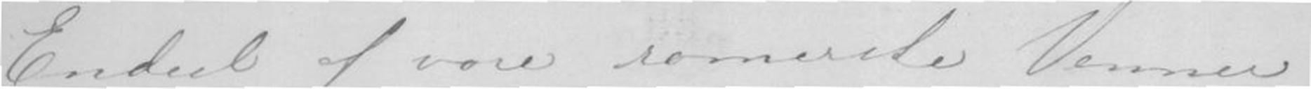Endeel af vore Venner
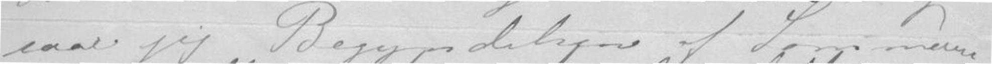jeg Begyndelsen af Sommeren
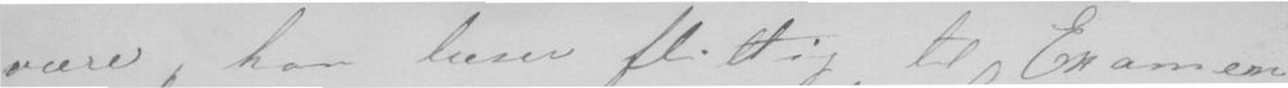være, han leser flittig til Examen
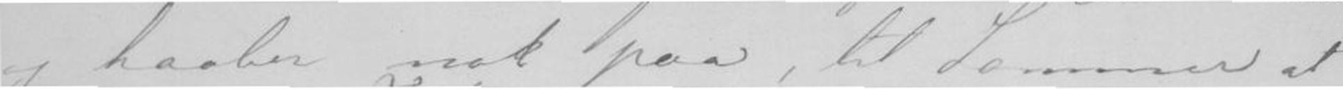og haaber nok paa, til Sommer at
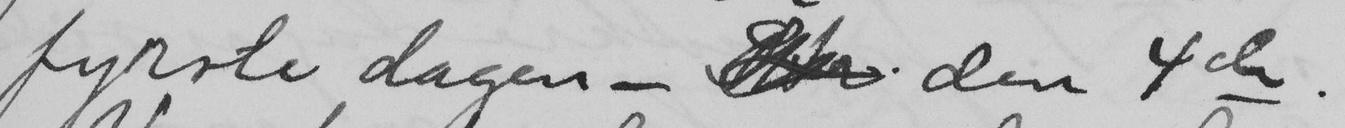fyrste dagen –  den 4de.
